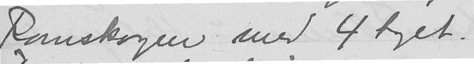Romskogen med 4 toget.
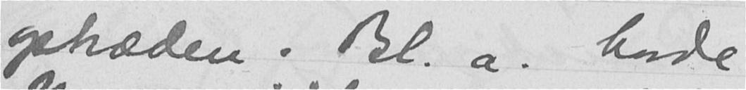optræden. Bl. a. havde
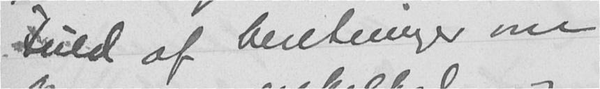Fuld af beretninger om
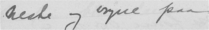heste og vogne paa
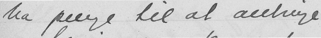ha penge til at anbringe
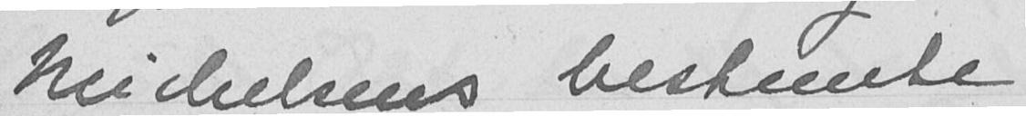Michelsens bestemte
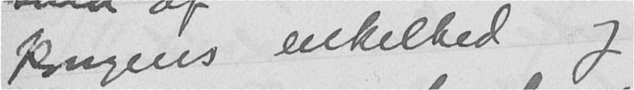kongens enkelhed og
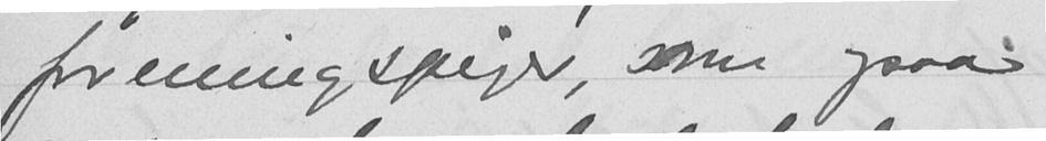foreningspiger, som ogsaa
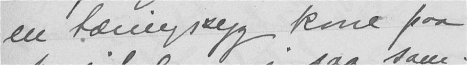en tæniegssyg kone paa
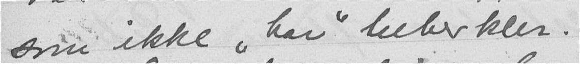som ikke "har" tuberkler.
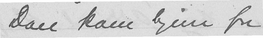Don kom hjem fra
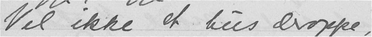Vel ikke et hus deroppe,
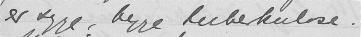er syge, begge tuberkulose.
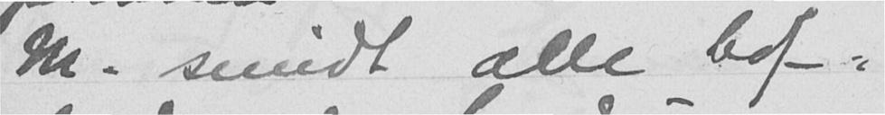M. smidt alle hof-
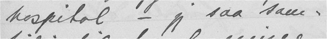hospital - jeg saa sam-
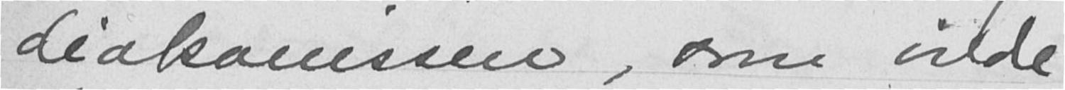diakonissen, som vilde
